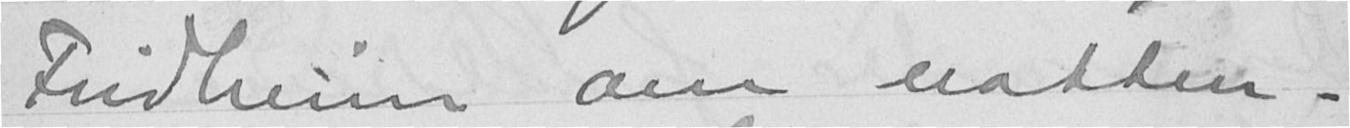Fridheim om natten.
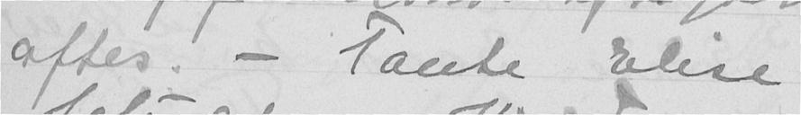aftes. - Tante Elise
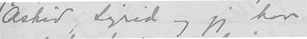Astrid, Sigrid og jeg har
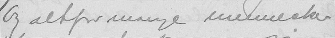Og altfor mange mennesker
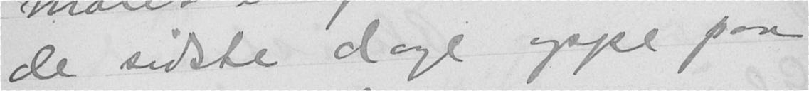de sidste dage oppe paa
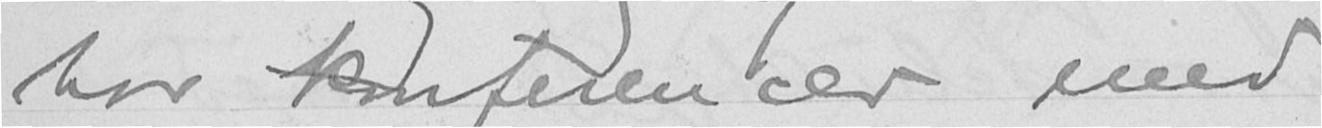har konferencer med
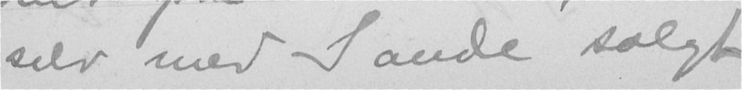selv med Sande solgt
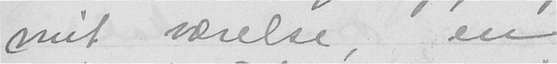mit værelse, en
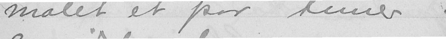malet et par timer
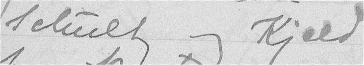Schultz og Kjeld
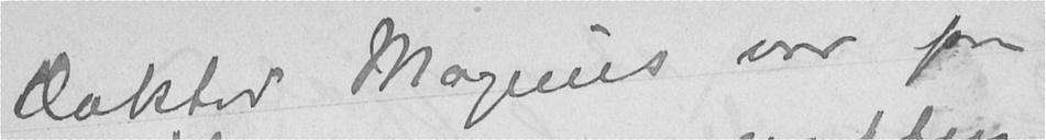Doktor Magnus var paa
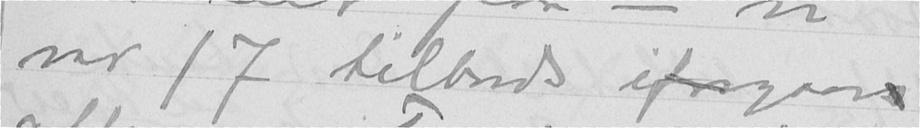var 17 tilbords iforgaars
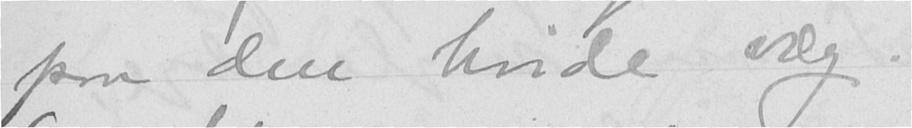paa den hvide væg.
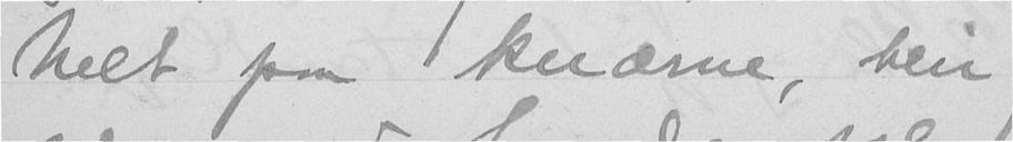helt paa knærne, blir
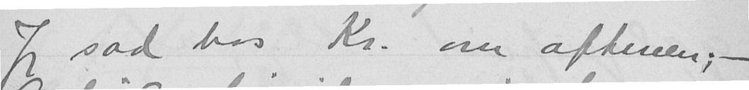Jeg sad hos Kr. om aftenen; -
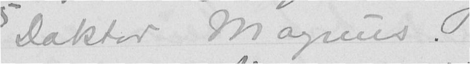Doktor Magnus.
